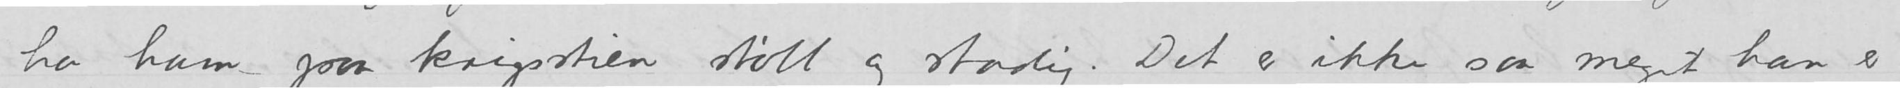ha ham paa krigsstien støtt og stadig. Det er ikke saa meget han er
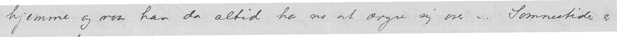hjemme og maa han da altid ha no at ærgre sig over –. Sommetider er
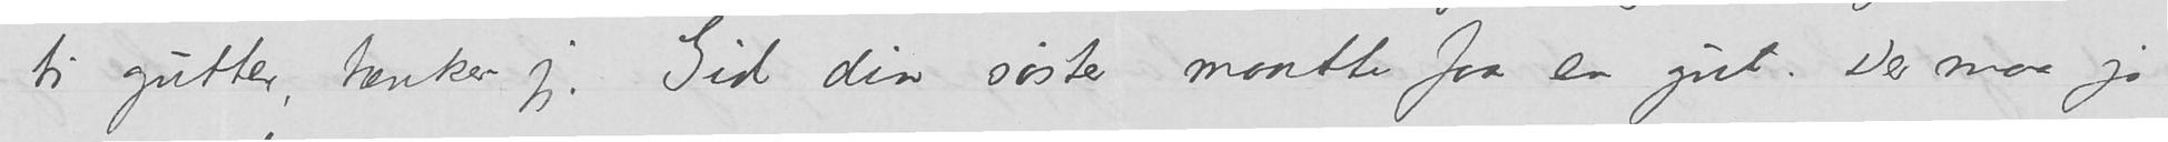ti gutter, tænker jeg. Gid din søster maatte faa en gut. Der maa jo
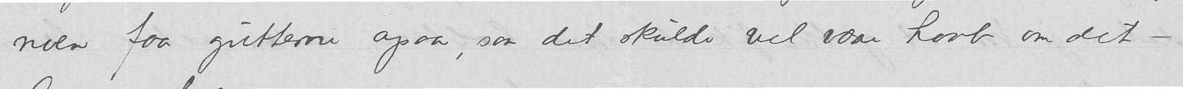noen faa gutterne ogsaa, saa det skulde vel være haab om det –.
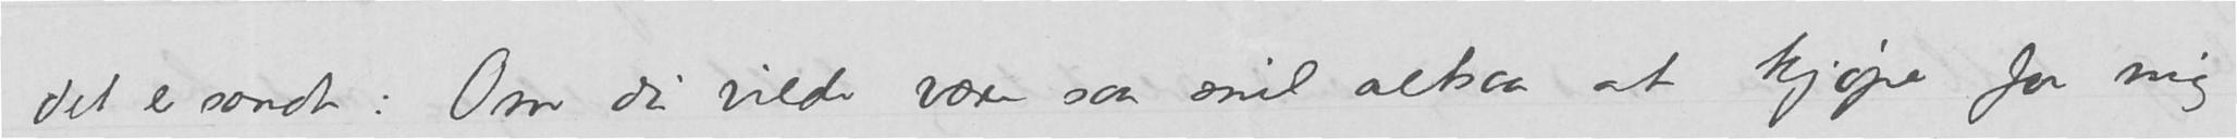Det er sandt: Om du vilde være saa snil altsaa at kjøpe for mig
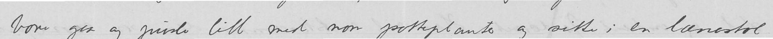bare gaa og pusle litt med non potteplanter og sitte i en lænestol
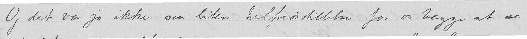Og det var jo ikke saa liten tilfredsstillelse for os begge at se
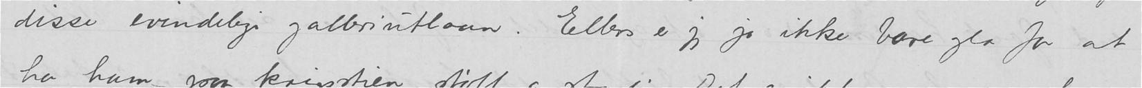disse evindelige galleriutlaan.  Ellers er jeg jo ikke bare gla for at
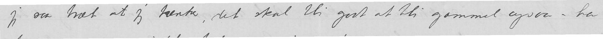jeg saa træt at jeg tænker, det skal bli godt at bli gammel ogsaa – ha
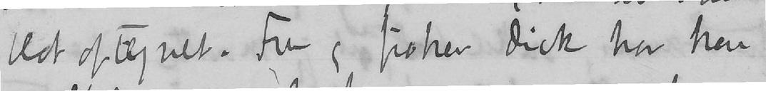blot optegnet. Fru og frøken Dick har han
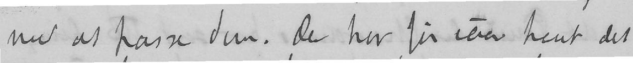med at passe dem. De har før iaar havt det
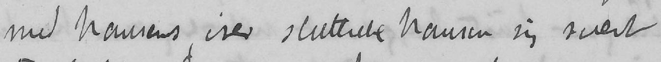med Nansens, især sluttede Nansen sig svært
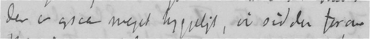Der er ogsaa meget hyggeligt, vi sidder foran
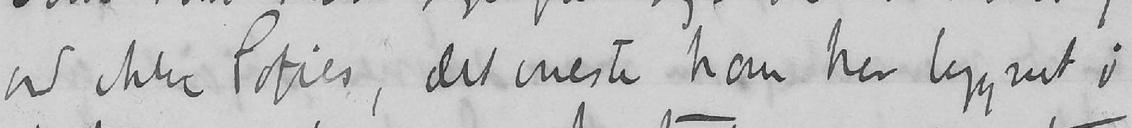ord ikke Sofies, det eneste han har begyndt i
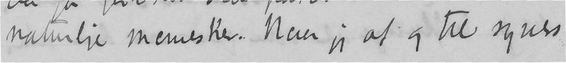naturlige mennesker. Naar jeg af og til synes
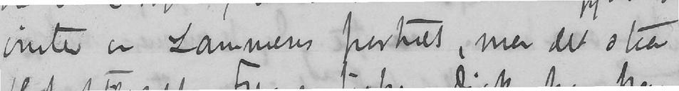vinter er Lammers portræt, men det staar
